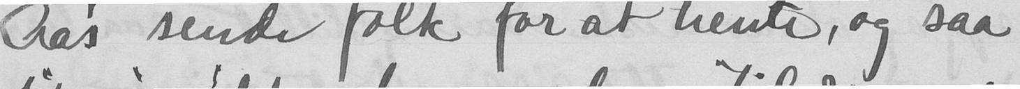Aas sende folk for at hente, og saa
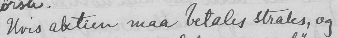Hvis aktien maa betales straks, og
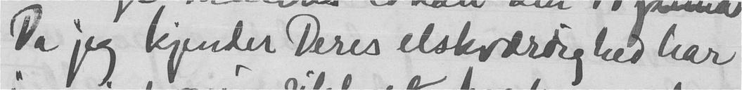Da jeg kjender Deres elskværdighed har
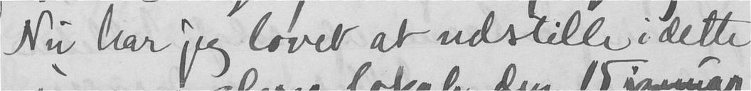Nu har jeg lovet at udstille i dette
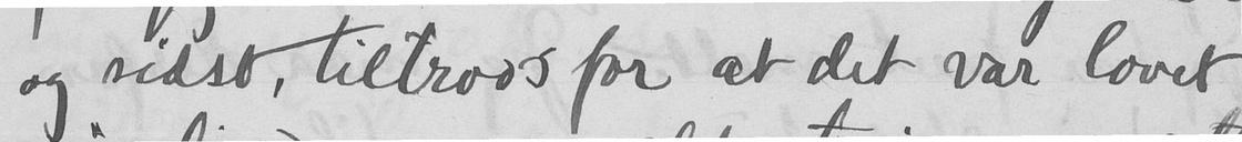og sidst tiltrods for at det var lovet
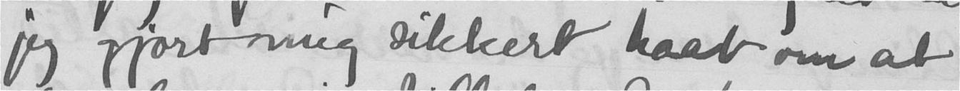jeg gjort mig sikkert haab om at
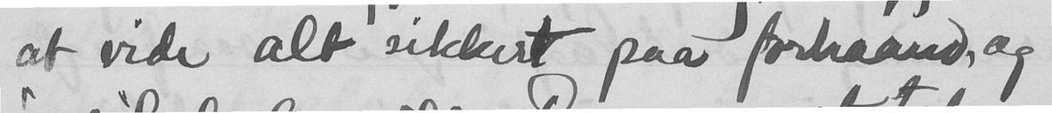at vide alt sikkert paa forhaand, og
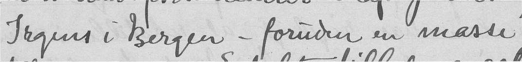Irgens i Bergen - foruden en masse
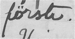første.
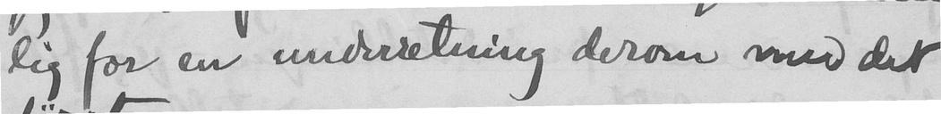lig for en underetning derom med det
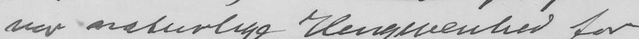vor naturlige Hengivenhed for
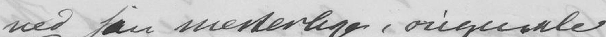ved sin mesterlige, origenale
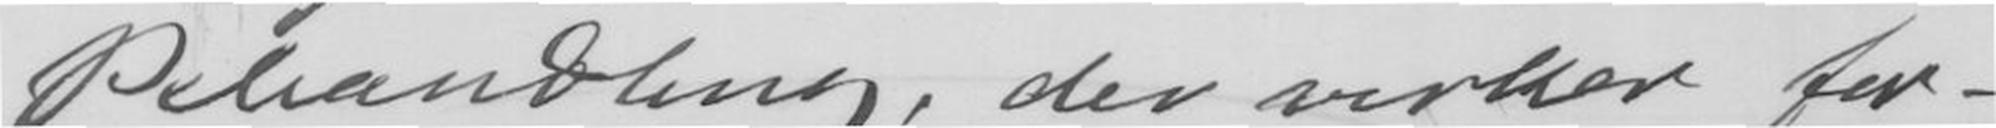Behandling, der virker for-
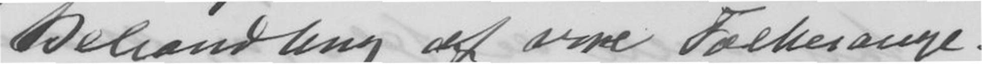Behandling af vore Folkesange.
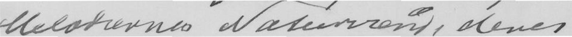Melodiernes Naturværd, Deres
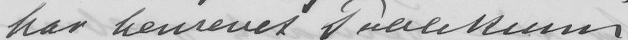har henrevet Publikum
